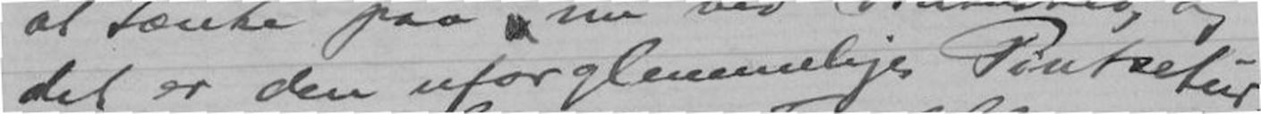det er den uforglemmelige Pintsetur!
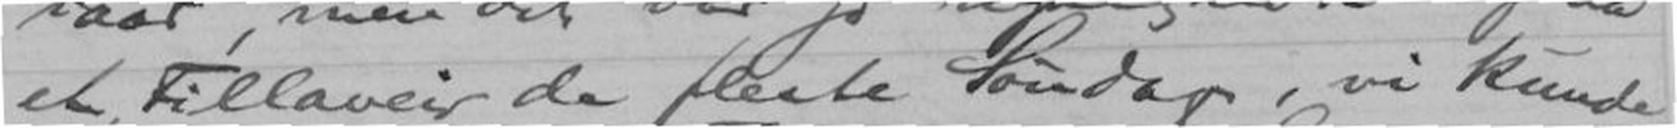et Fillaveir de fleste Søndage, vi kunde
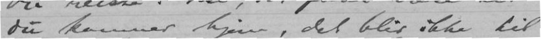Du kommer hjem, det blir ikke til
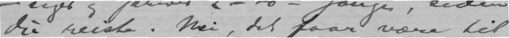Du reiste. Nei, det faar være til
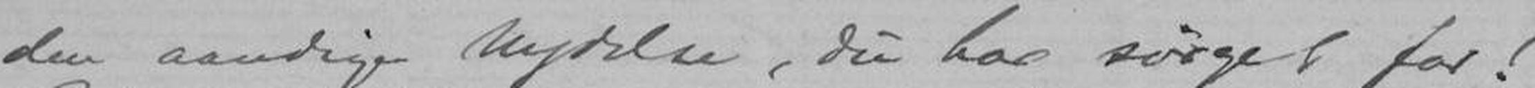den aandige Nydelse, Du har sørget for!
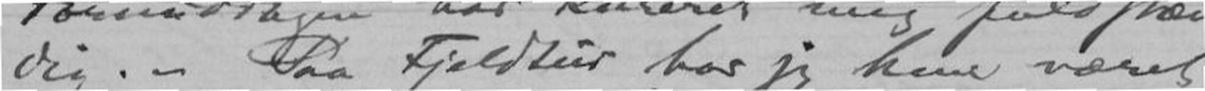dig. - Paa Fjeldtur har jeg kun været
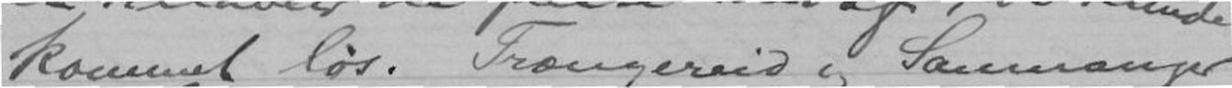kommet løs. Trængereid og Samnanger
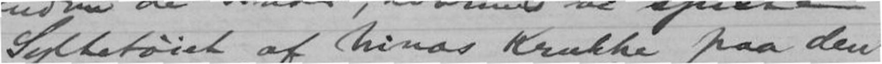Syltetøiet af Ninas Krukke paa den
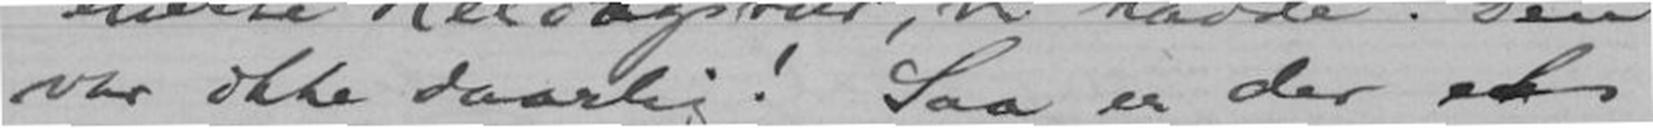var ikke daarlig! Saa er der et
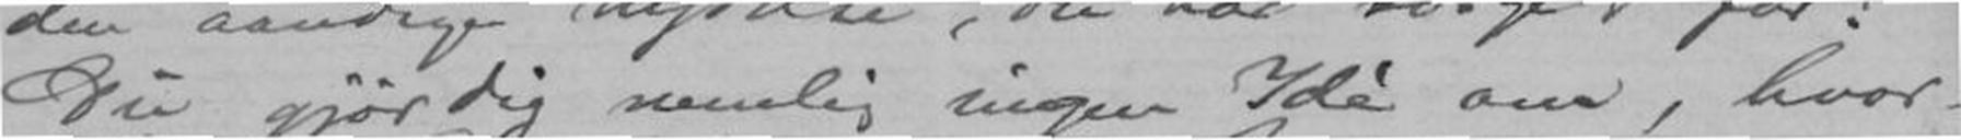Du gjør dig nemlig ingen Idè om, hvor-
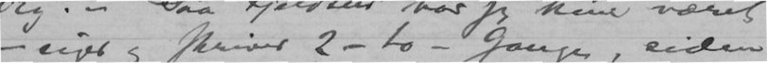- siger og skriver 2 - to- Gange, siden
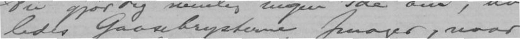ledes Gaasebrysterne smager, naar
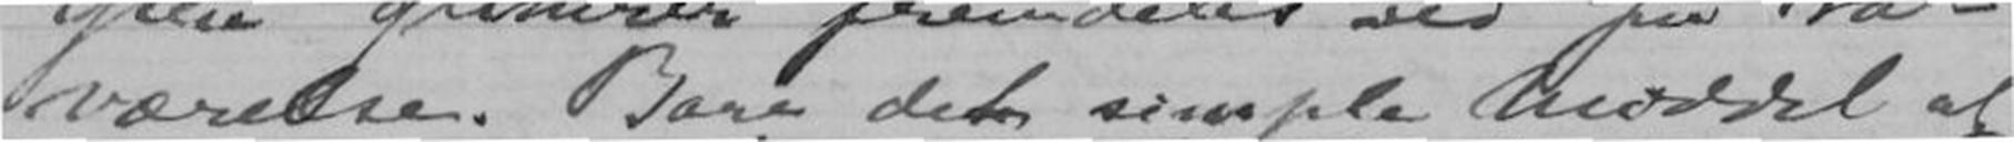værelse. Bare det simple Middel at
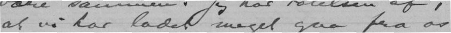at vi har ladet meget gaa fra os
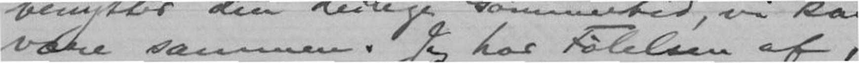være sammen. Jeg har Følelsen af,
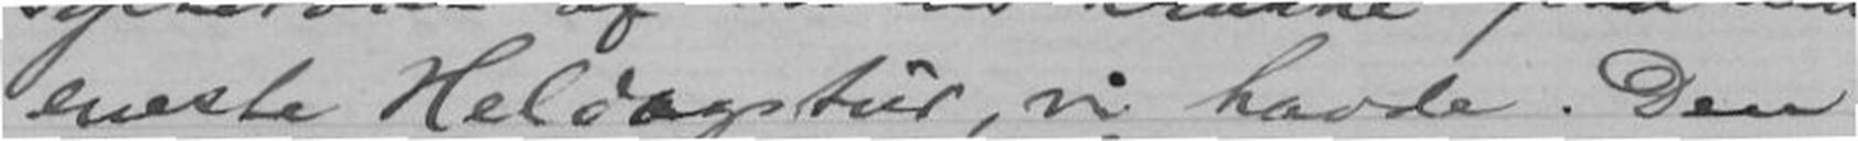eneste Heldagstur, vi havde. Den
